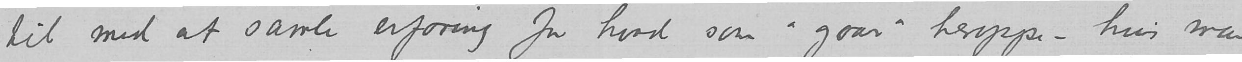til med at samle erfaring for hvad som «gaar» heroppe – hvis man
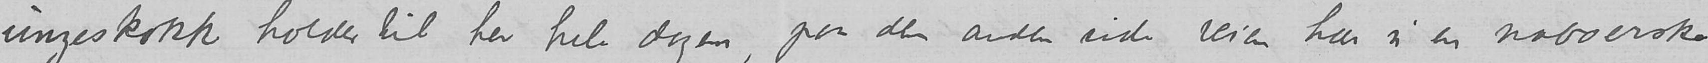ungeskokk holder til her hele dagen, paa den anden side veien har vi en naboerske
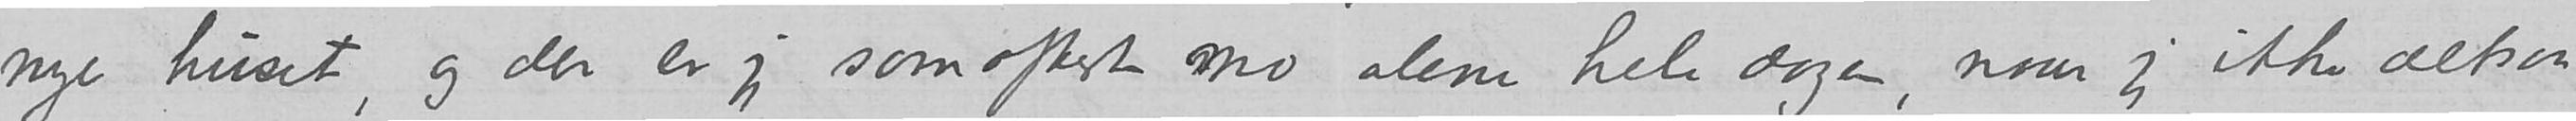nye huset, og der er jeg somoftest mo alene hele dagen, naar jeg ikke altsaa
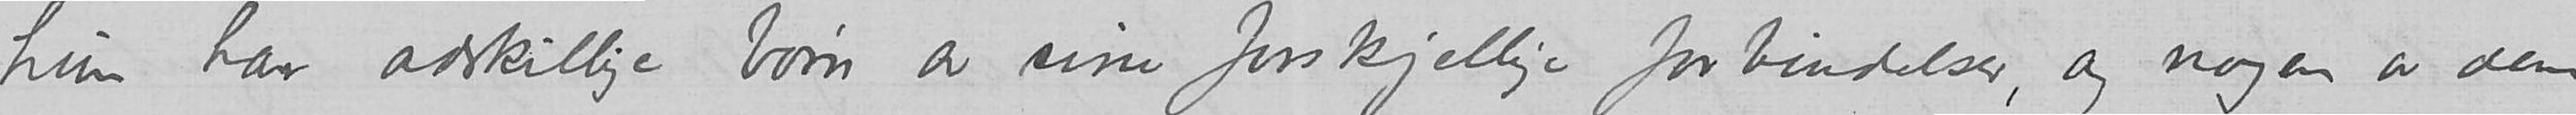hun har adskillige børn av sine forskjellige forbindelser, og nogen av dem
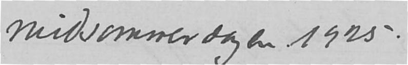midsommerdagen 1925.
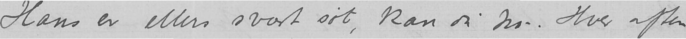Hans er ellers svært søt, kan du tro –. Hver aften
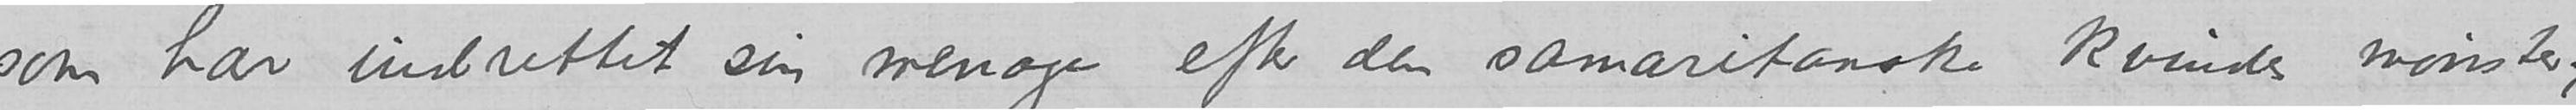som har indrettet sin menage efter den samaritanske kvindes mønster;
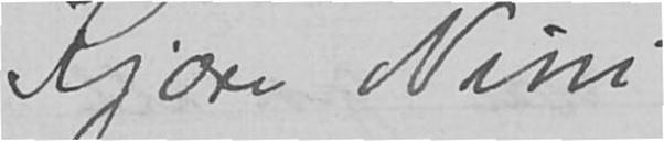Kjære Nini,
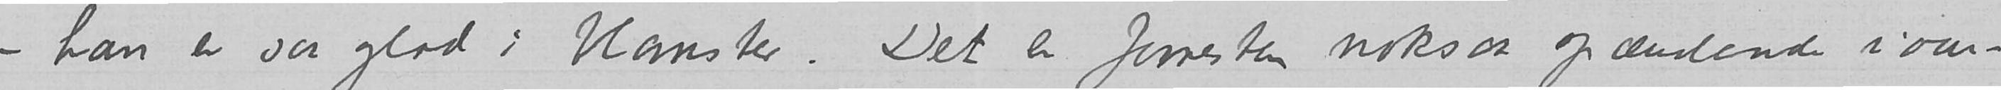han er saa glad blomster. Det er forresten noksaa spændende iaar –
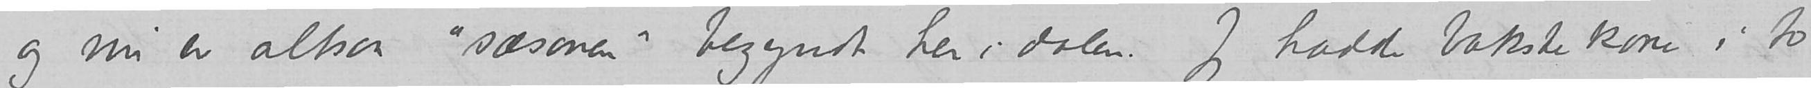og nu er altsaa «sæsonen» begyndt her i dalen. Jeg hadde bakstekone i to
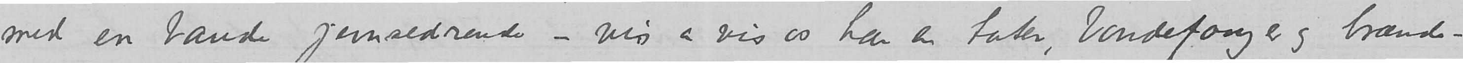med en bande jevnaldrende – vis a vis os har en tater, bondefanger og brænde-
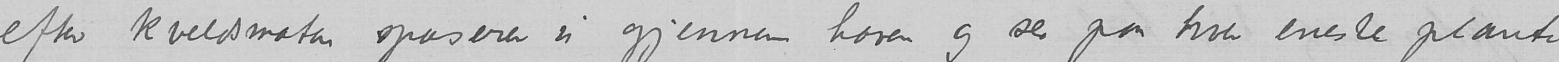efter kveldsmaten spaserer vi gjennem haven og ser paa hver eneste plante –
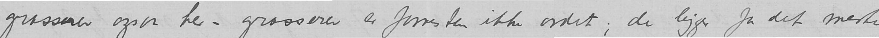grasserer ogsaa her – grasserer er forresten ikke ordet; de ligger for det meste
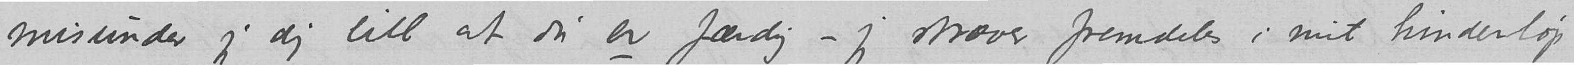misunder jeg dig litt at du er færdig – jeg stræver fremdeles i mit hinderløp,
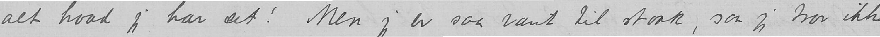selv alt hvad jeg har set! Men jeg er saa vant til staak, saa jeg tror ikke
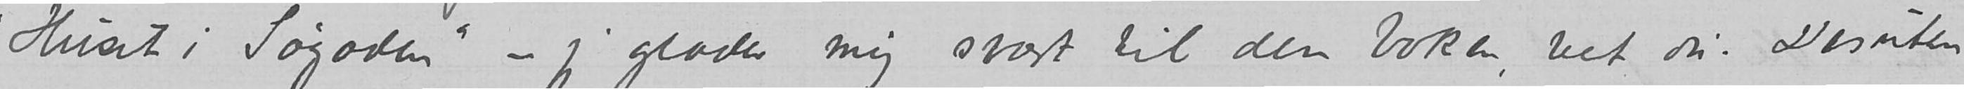«Huset i Sögaden» – jeg glæder mig svært til den boken, vet du. Desuten
